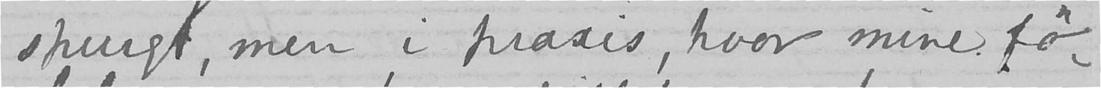spurgt, men i praxis, hvor mine fø-
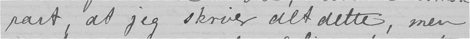rart, at jeg skriver alt dette, men
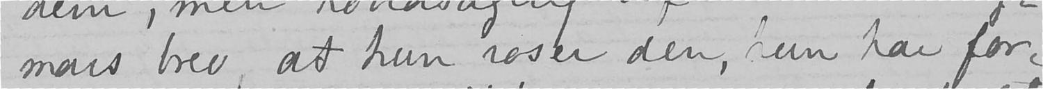mars brev, at hun roser den, hun har for-
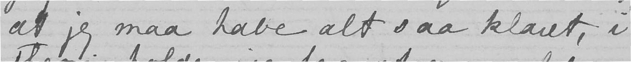at jeg maa have alt saa klaret, i
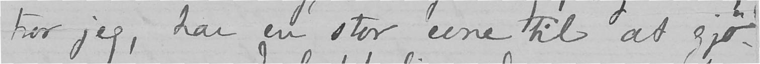tror jeg, har en stor evne til at gjø-
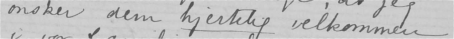ønsker dem hjertelig velkommen
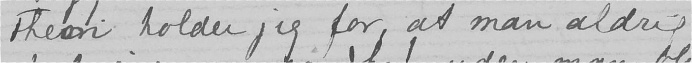theori holder jeg for, at man aldrig
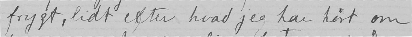frygt, lidt efter hvad jeg har hørt om
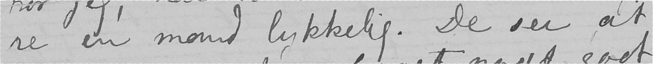re en mand lykkelig. De ser at
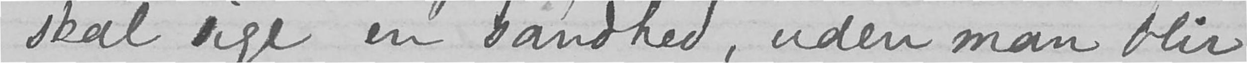skal sige en sandhed, uden man blir
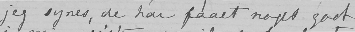jeg synes, de har faaet noget godt
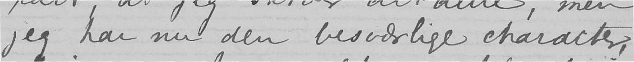jeg har nu den besværlige character,
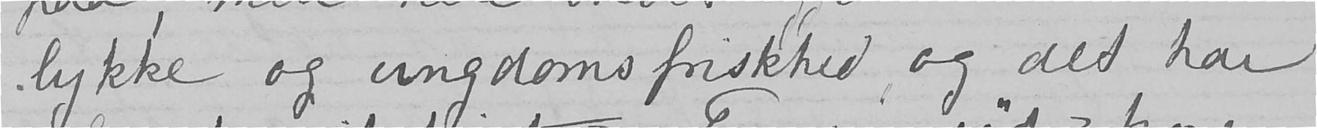lykke og ungdomsfriskhed, og det har
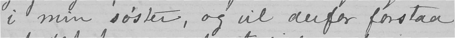i min søster, og vil derfor forstaa
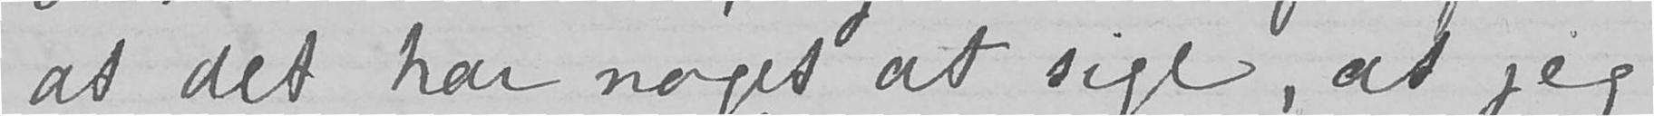at det har noget at sige, at jeg
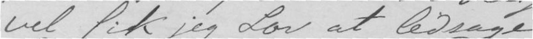vel fik jeg Lov at ledsage
